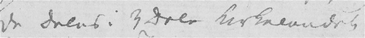de deles i 3 Dele Kirkelandet
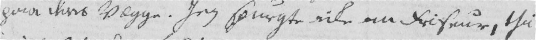paa deres Vægge. Jeg spurgte icke om Friseur, thi
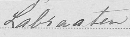Labraaten
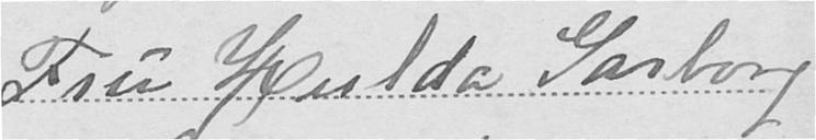Fru Hulda Garborg
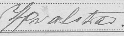Hvalstad.
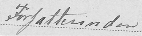Forfatterinden
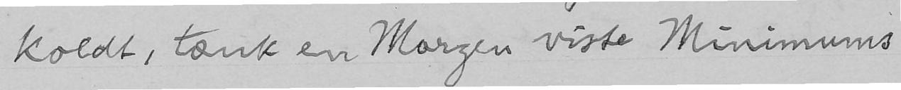koldt, tænk en Morgen viste Minimums
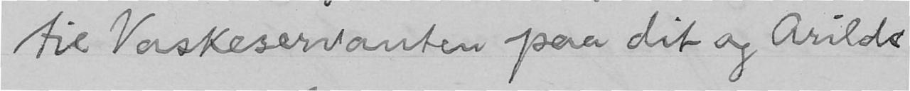til Vaskeservanten paa dit og Arilds
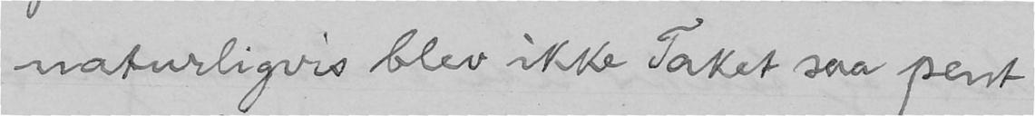naturligvis blev ikke Taket saa pent
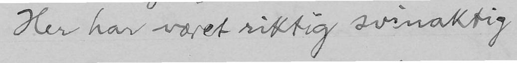Her har været riktig svinaktig
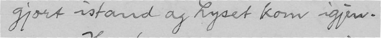gjort istand og Lyset kom igjen.
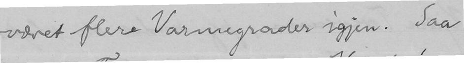været flere Varmegrader igjen. Saa
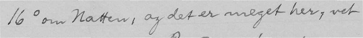16˚ om Natten, og det er meget her, vet
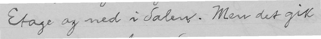Etage og ned i Salen. Men det gik
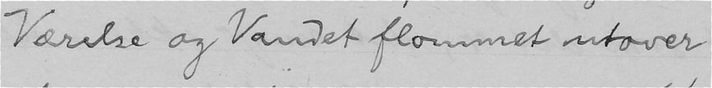Værelse og Vandet flommet utover
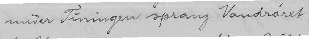under Tiningen sprang Vandrøret
